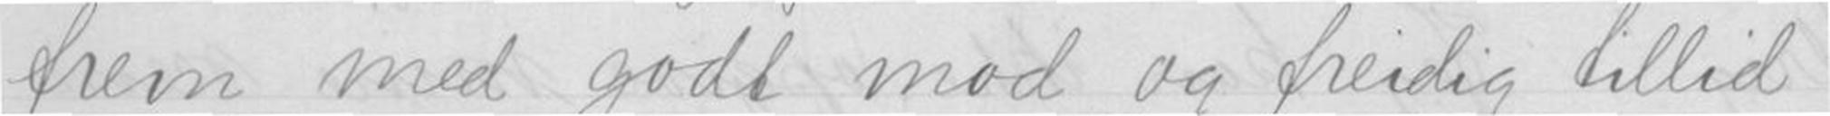frem med godt mod og freidig tillid.
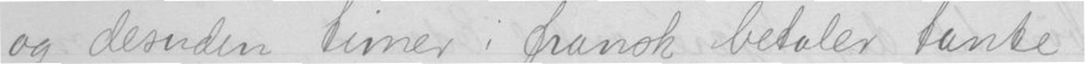og desuden tiner i fransk betaler tante
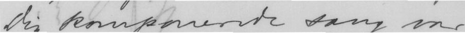Dig komponerede sang var
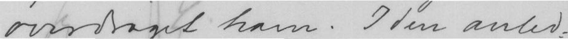overdraget ham. Iden anled-
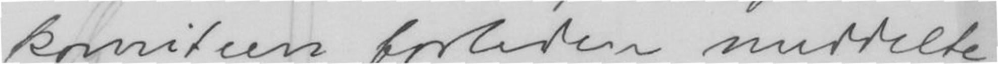komiteen forleden meddelte
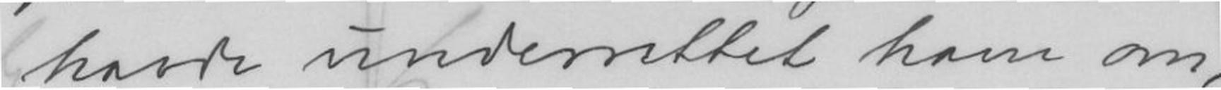havde underrettet ham om,
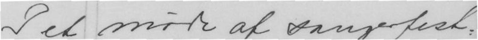I et møde af sangerfest-
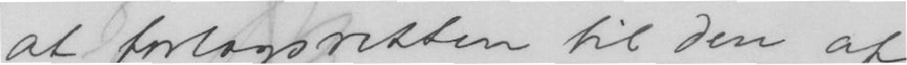ag forlagsretten til den af
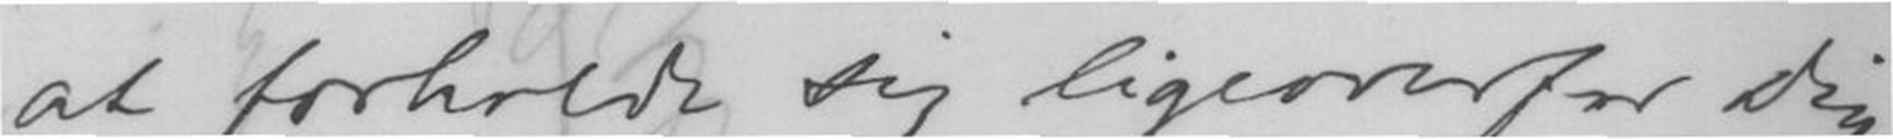at forholde sig lige ovenfor dig.
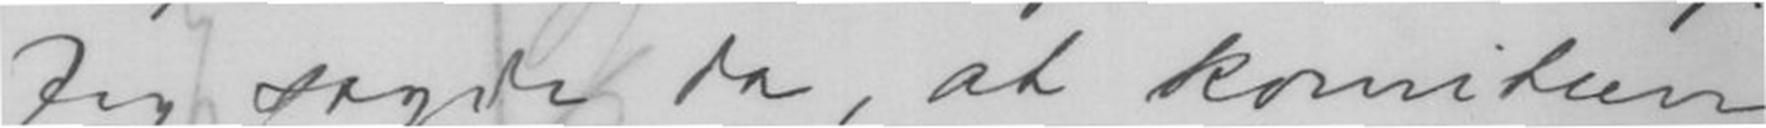Jeg sagde da, at komiteen
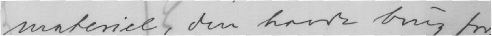materiel, den havde brug for,
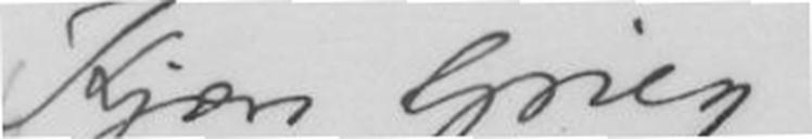Kjære Grieg
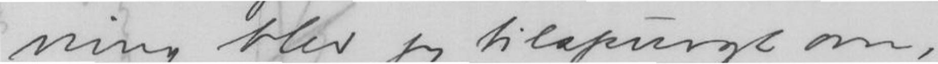ning blev jeg tilspurgt om,
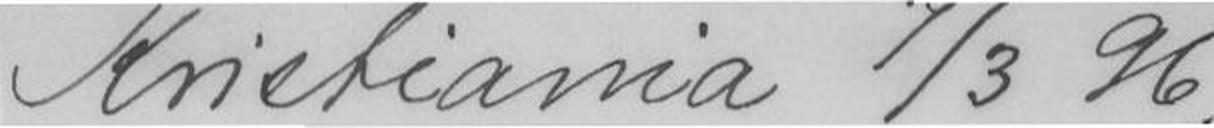Kristiania 7/3 96
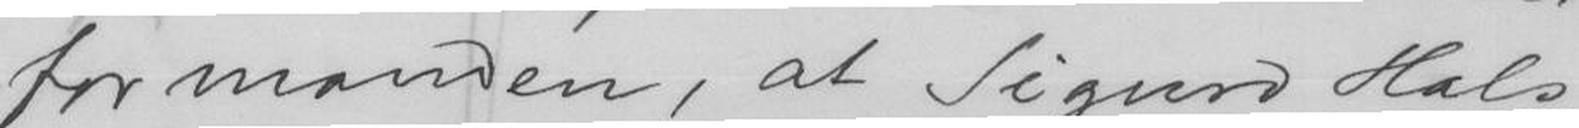formanden, at Sigurd Hals
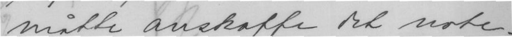måtte anskaffe det note-
